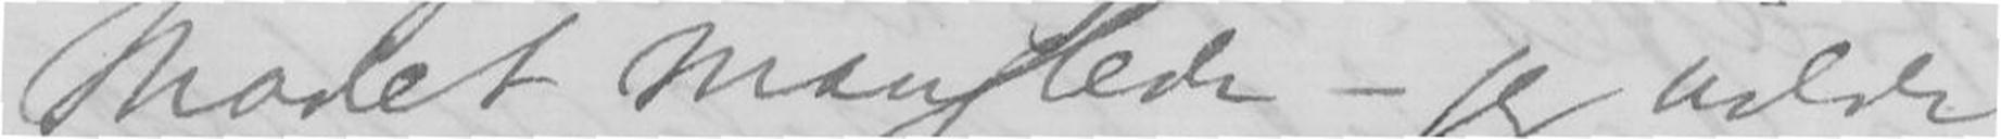Modet manglede - jeg vilde
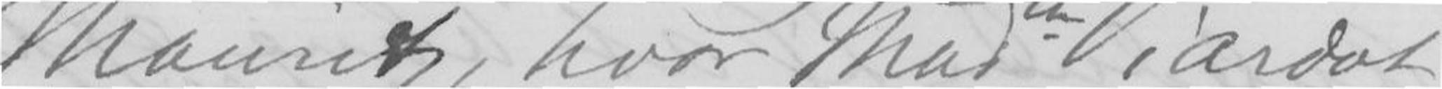Mauritz hvor Madm Viardot
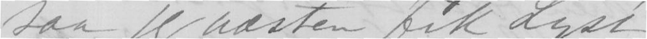saa jeg næsten fik Lyst
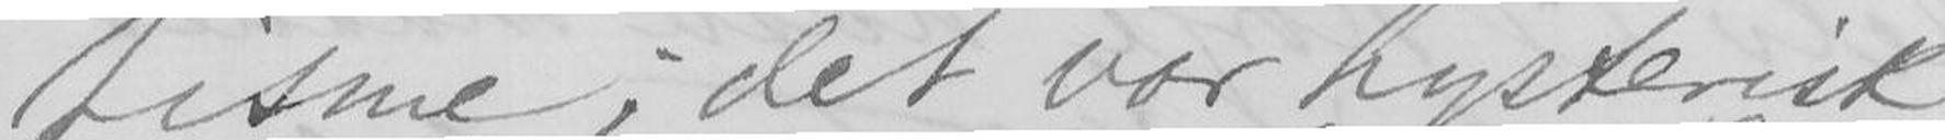-tisme; det var hysteresk,
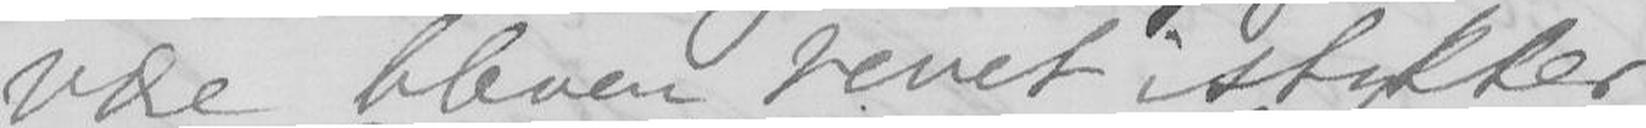være bleven revet istykker.
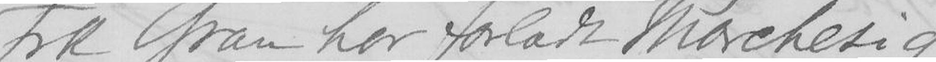Frk Gran har forladt Morchesig
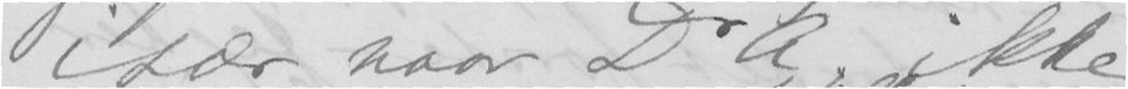især naar D A ikke
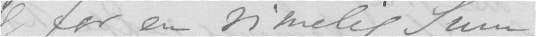og for en rimelig Sum,
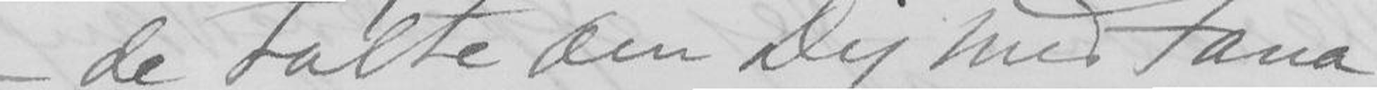- de talte om Dig med Fana
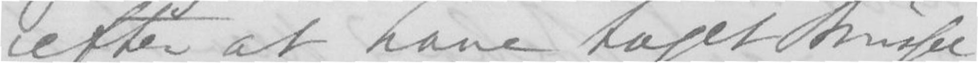efter at have taget Brussel.
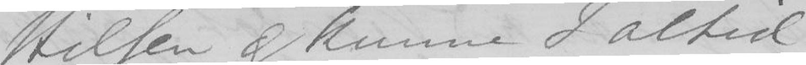Hilsen og kunne I altid
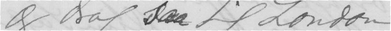og drag saa til London. -
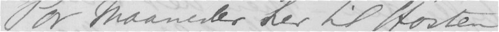Par Maaneder her til Høsten
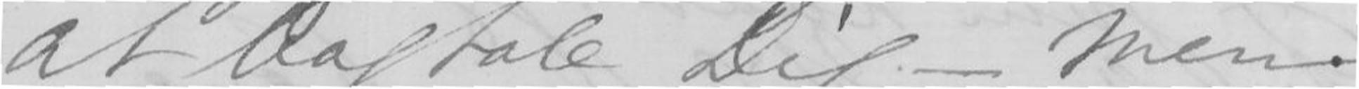at bagtale Dig - Men
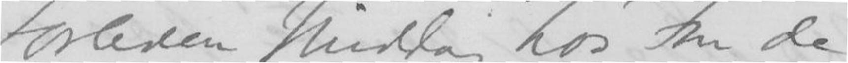Forleden Middag hos Fru de
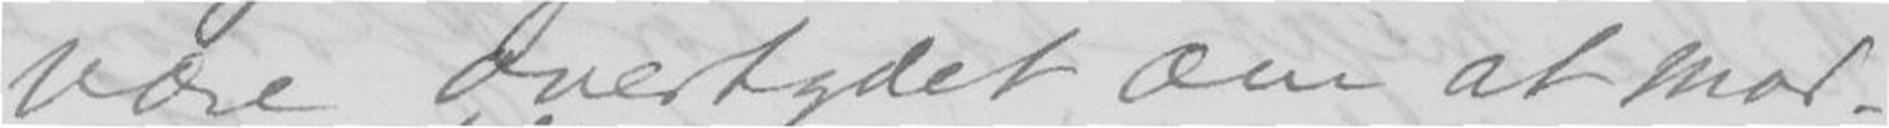være overtydet om at mod-
